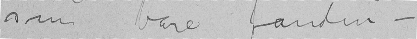som bare fanden –
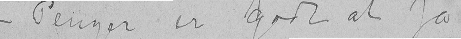– Penger er godt at få
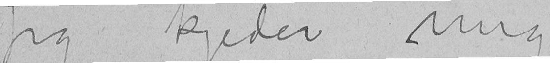Jeg kjeder mig
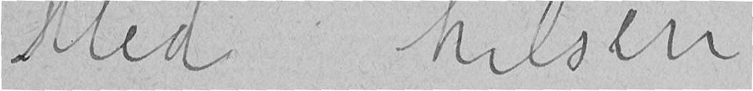Med hilsen
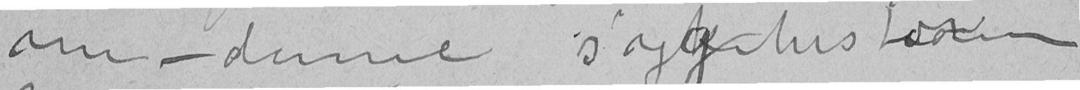nu – denne sygehistorien
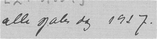alle sjæles dag 1927.
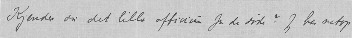Kjender du det lille officium for de døde? Jeg har netop
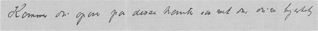Kommer du opover paa disse kanter saa vet du, du er hjertelig
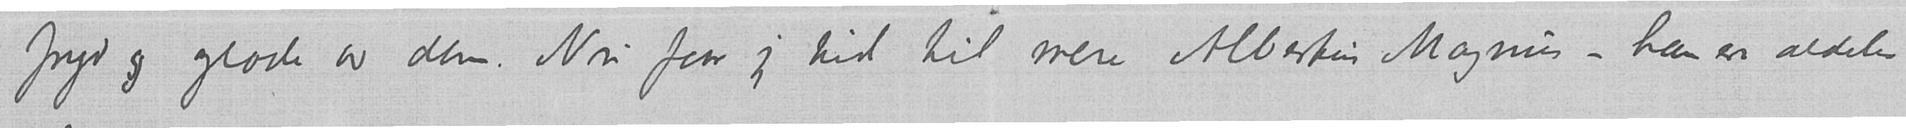fryd og glæde av dem. Nu faar jeg tid til mere Albertus Magnus – han er aldeles
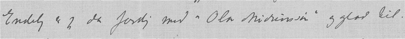Endelig er jeg da færdig med "Olav Audunssøn" og glad til.
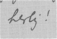herlig!
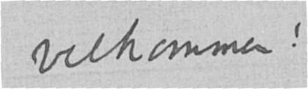velkommen!
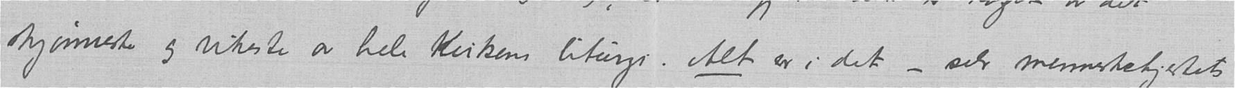skjønneste og rikeste av hele Kirkens liturgi.  Alt er i det – selv menneskehjertets
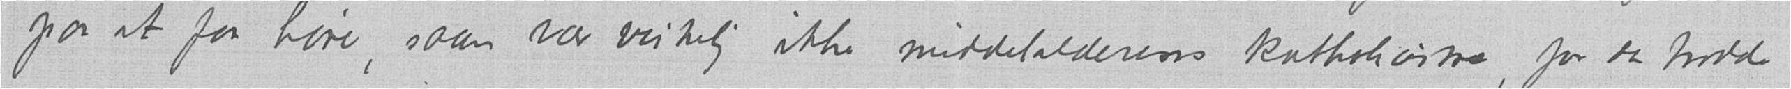paa at faa høre, saan var virkelig ikke middelalderens katholicisme, for da trodde
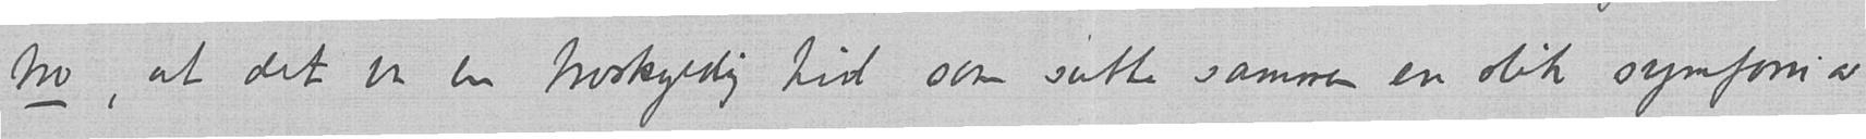tro, at det var en troskyldig tid som satte sammen en slik symfoni av
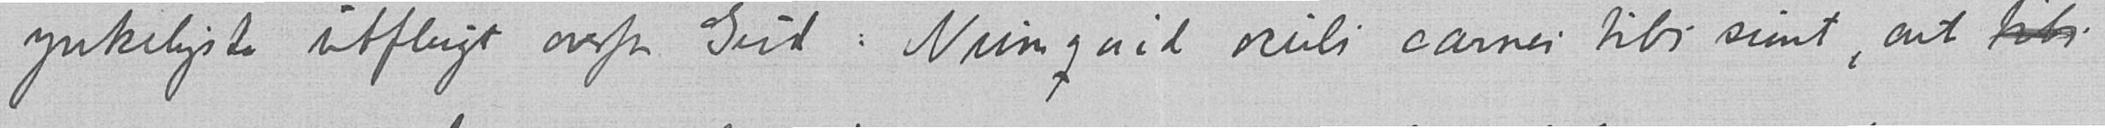ynkeligste utflugt overfor Gud: Numquid oculi carnei tibi sunt, aut tøbs.
